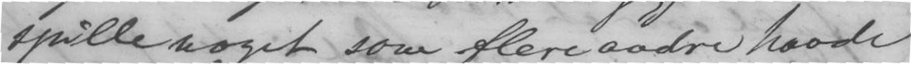spille noget som flere andre havde
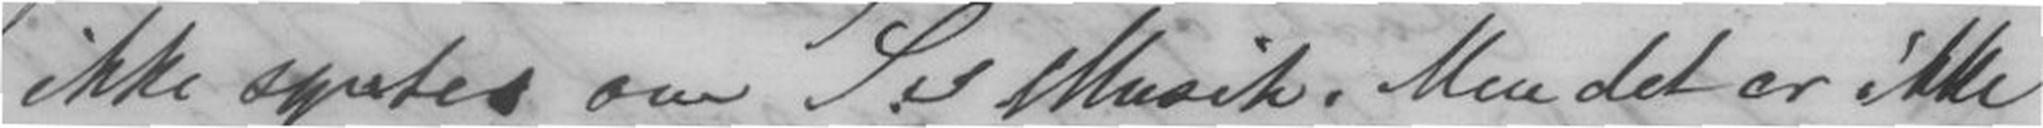ikke syntes om S's Musik. Men det er ikke
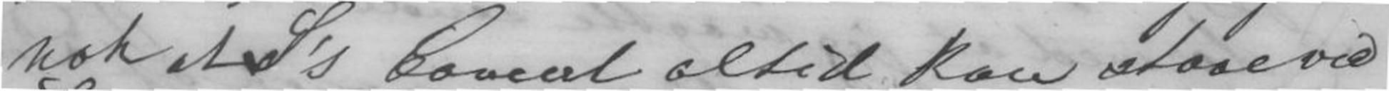nok at S's Concert altid kan staae ved
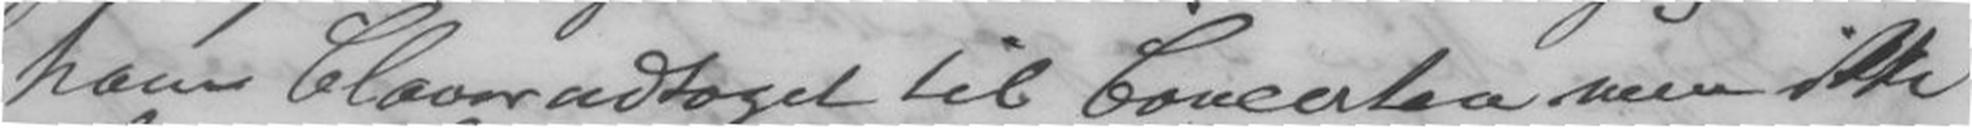hans Claver udtaget til Concerten men ikke
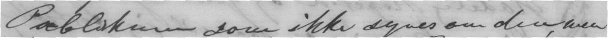Publikum som ikke synes om den, men
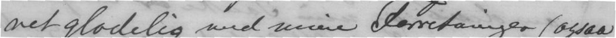ret glædelig med mine Forretninger (ogsaa
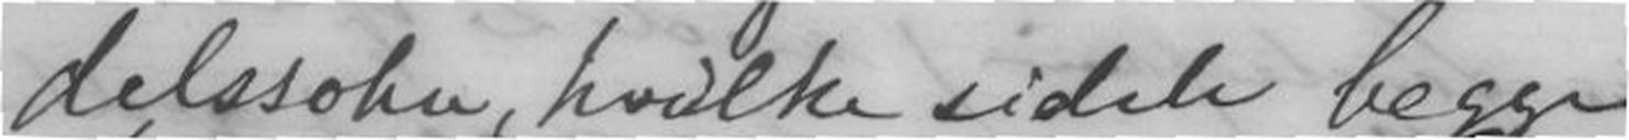delssohn hvilke sidste begge
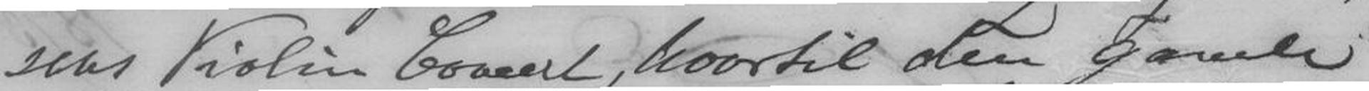sens Violin Concert, hvortil den gamle
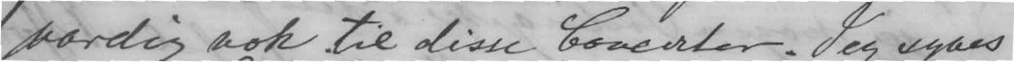værdig nok til disse Concerter. Jeg synes
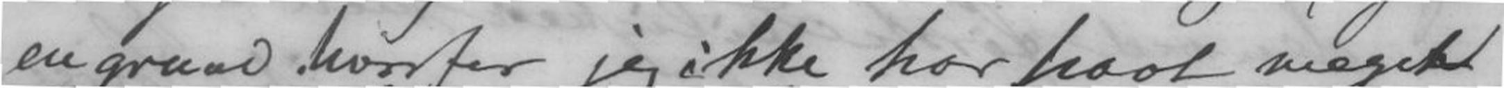en grund hvorfor jeg ikke har havt meget
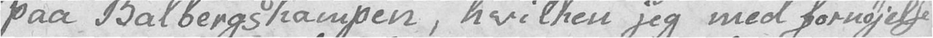paa Balbergskampen, hvilken jeg med fornøjelse
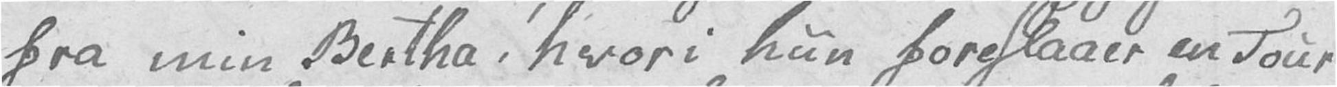fra min Bertha! hvori hun foreslaaer en Tour
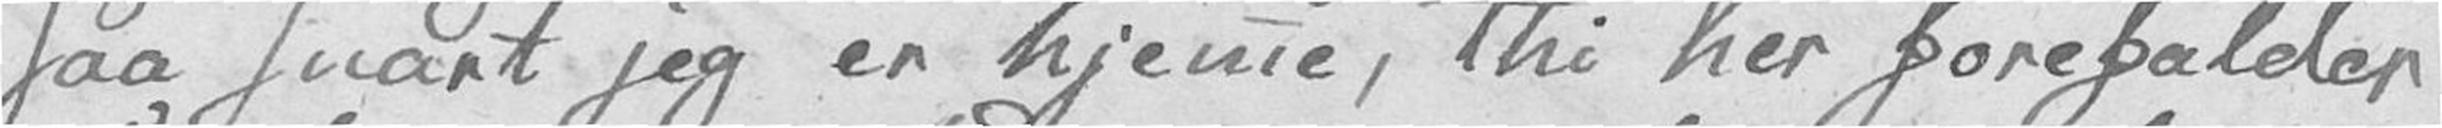saa snart jeg er hjemme, thi her forefalder
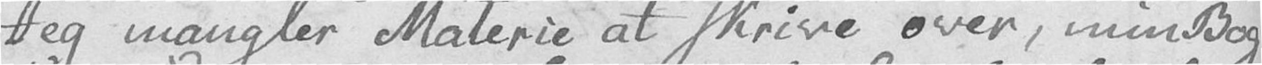Jeg mangler Materie at skrive over, min Bog
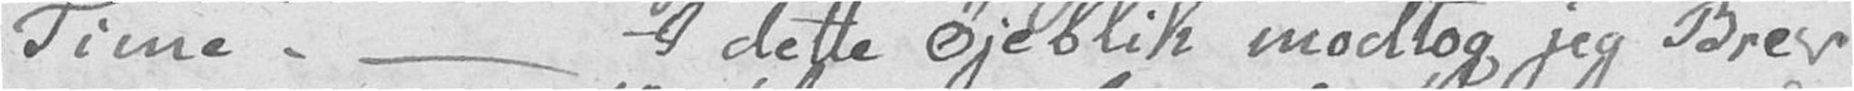Time. – I dette Øjeblik modtog jeg Brev
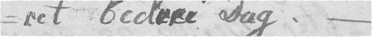-ret bedre i Dag. –
